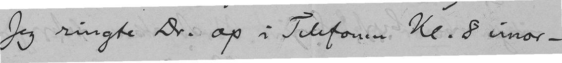Jeg ringte Dr. op i Telefonen Kl. 8 imor-
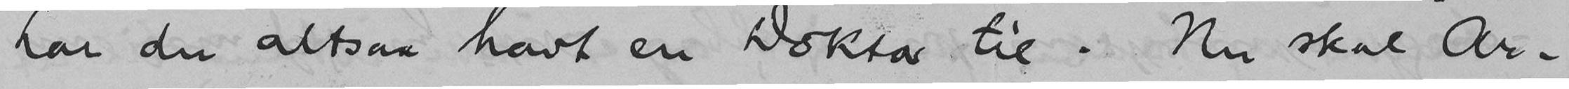har du altsaa havt en Doktor til. Nu skal Ar-
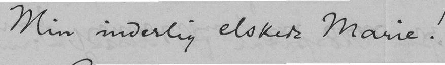Min inderlig elskede Marie!
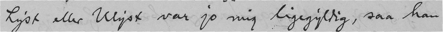Lyst eller Ulyst var jo mig ligegyldig, saa han
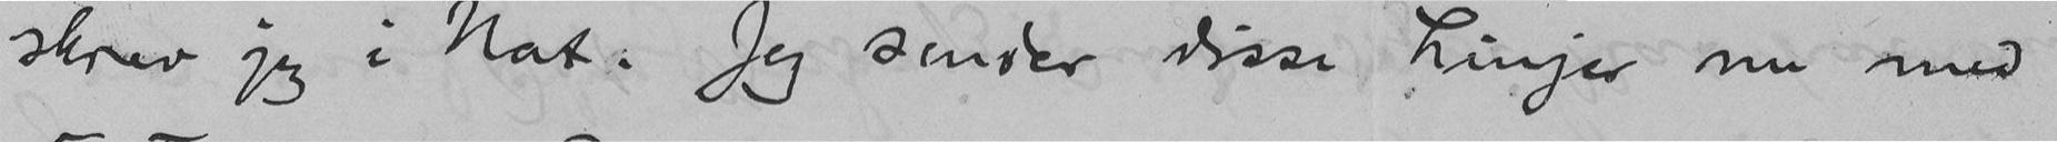skrev jeg i Nat. Jeg sender disse Linjer nu med
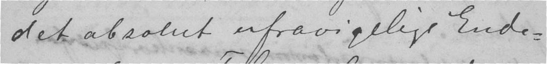det absolut ufravigelige Ende-
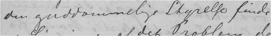den guddommelige Styrelse finde
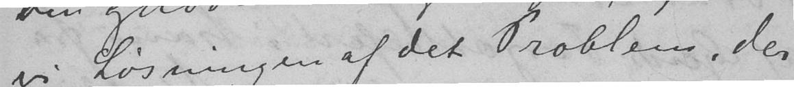vi Løsningen af det Problem, der
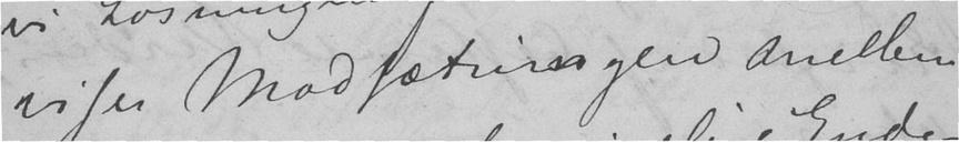viser Modsætningen mellem
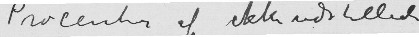Procenter af ikke udstillede
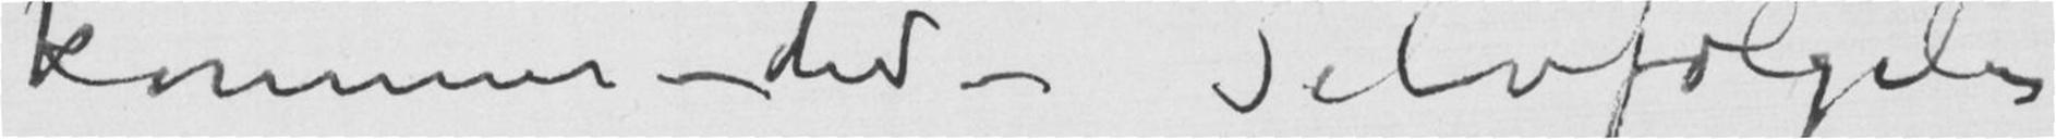kommer – did – Selvfølgeli
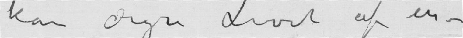kan ærgre Livet af en –
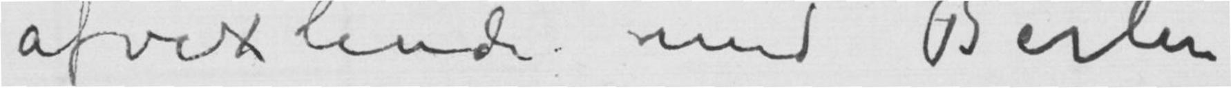afvexlende med Berlin
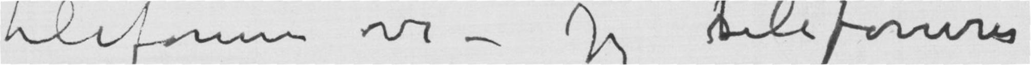telefonerer vi – Jeg stelefonerer
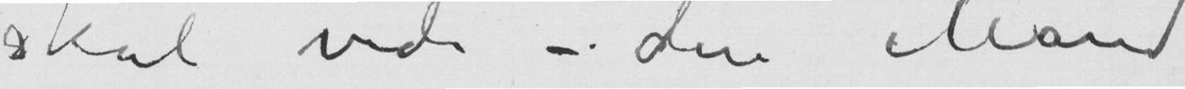skal vide – den Mand
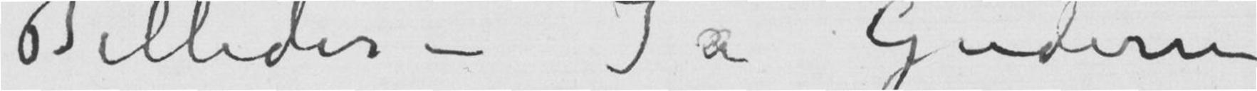Billeder – Ja Guderne
